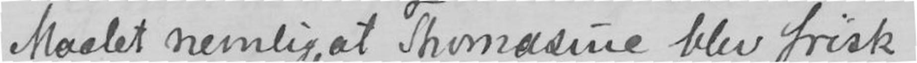Maalet nemlig, at Thomasine blev frisk.
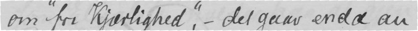om "for Kjærlighed", - det gaar enda an
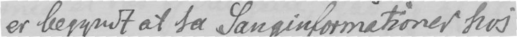er begyndt at ta Sanginformationer hos
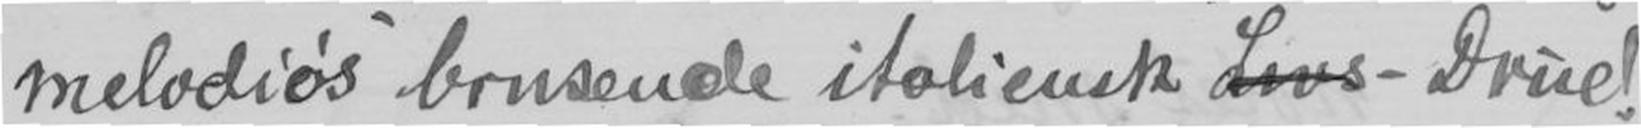melodiøs brusende italiensk - Drue!
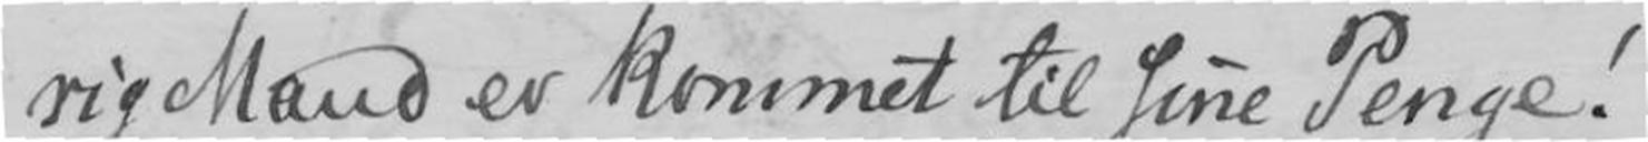rig Mand er kommet til sine Penge!
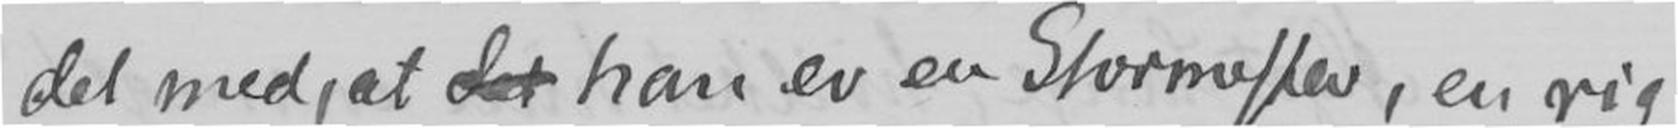det med, at det han er en Stormester, en rig
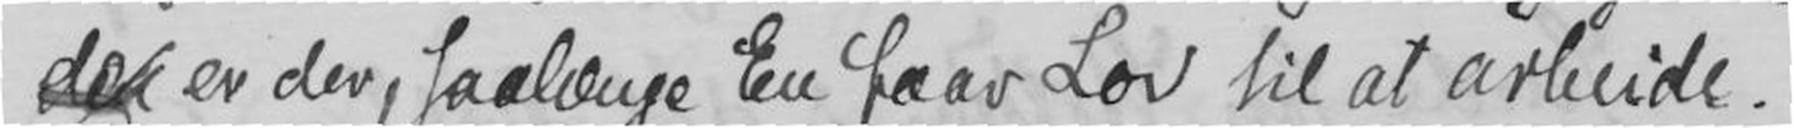er der, salænge En faar Lov til at arbeide.
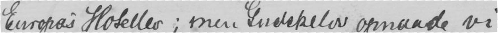Europas Hoteller; men Gudskelov opnaade vi
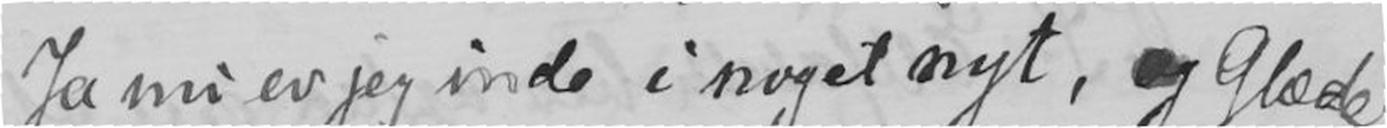Ja nu er jeg inde i noget nyt, og Glæde
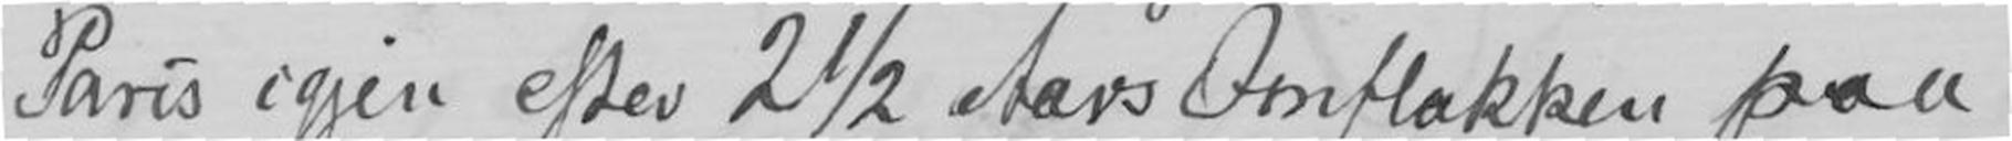Paris igjen efter 2½ Aars Omflakken paa
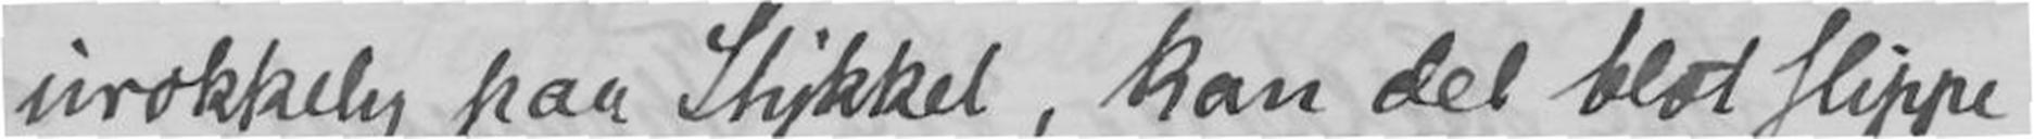urokkelig paa Stykket, kan det blot slippe
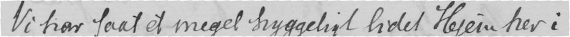Vi har faat et meget hyggeligt lidet Hjem her i
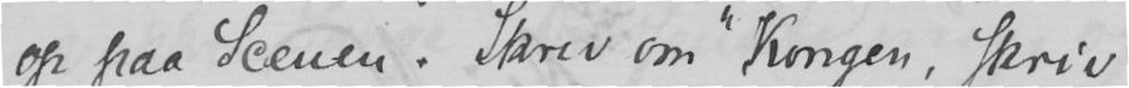op paa Scenen. Skrev om " Kongen, skriv
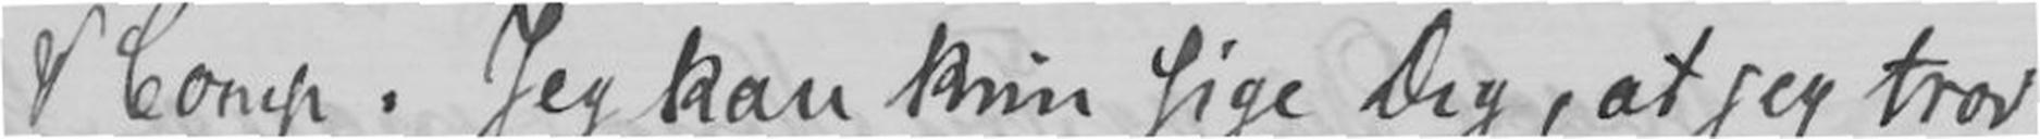& Comp. Jeg kan kun sige Dig, at jeg tror
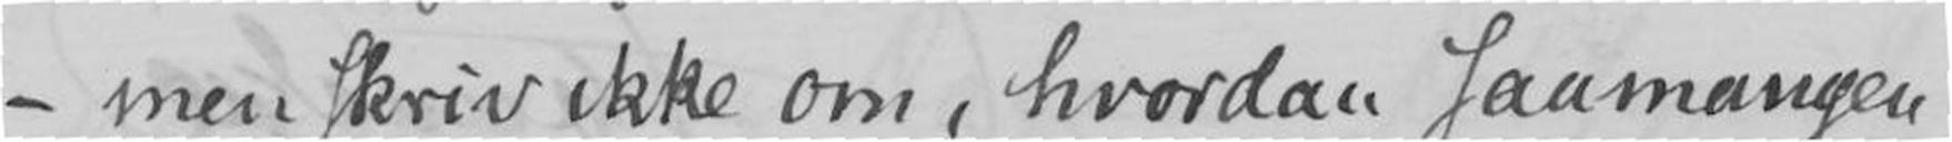- men skriv ikke om, hvordan saamangen
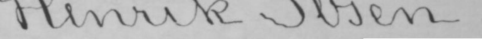Henrik Ibsen.
